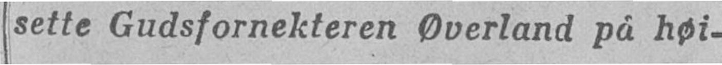sette Gudsfornekteren Øverland på høi-
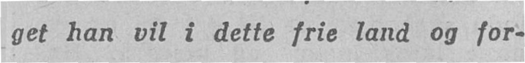get han vil i dette frie land og for-
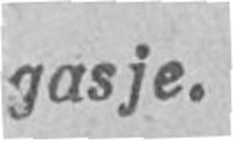gasje.
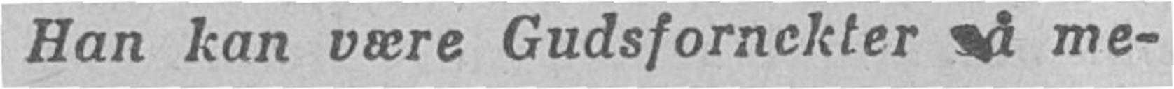Han kan være Gudsfornekter så me-
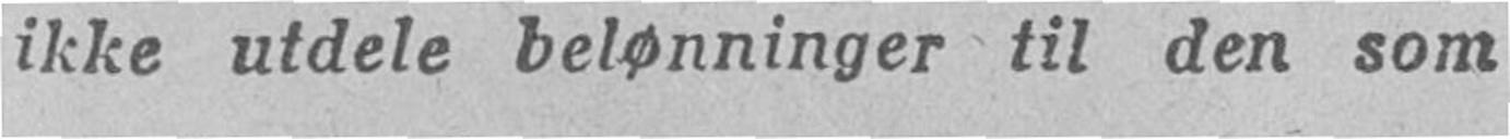ikke utdele belønninger til den som
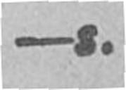—s.
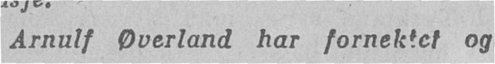Arnulf Øverland har fornektet og
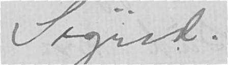Sigrid.
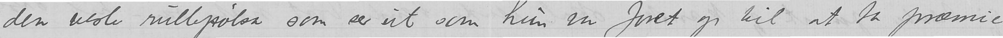den vesle rullepølsa som ser ut som hun var foret op til at ta præmie
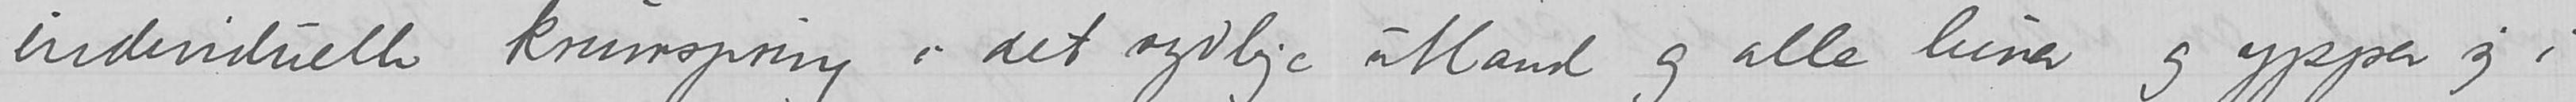individuelle krumspring i det sydlige utland og alle luner og ypper sig i
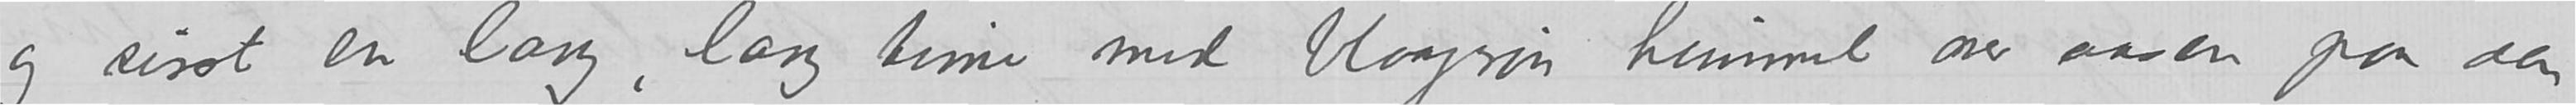og sisst en lang, lang time med blaagrøn himmel over aasen paa den
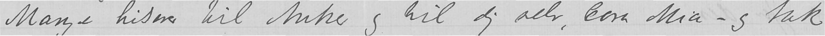Mange hilsener til Anker og til dig selv, Cora Mia – og tak
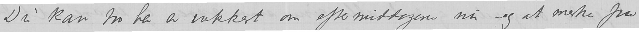Du kan tro her er vakkert om eftermiddagen nå – og at merke fra
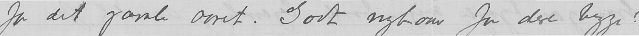for det gamle aaret. Godt nytaar for dere begge.
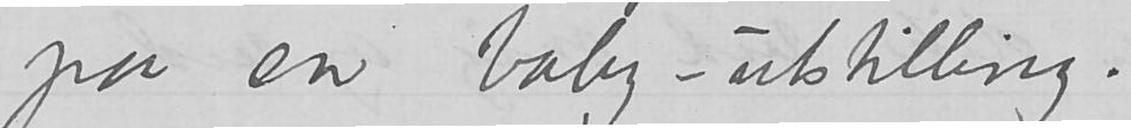paa en baby-utstilling.
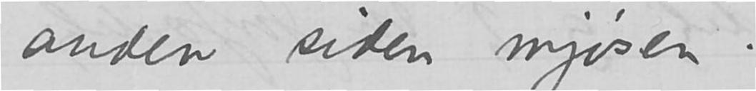anden siden mjøsen!
